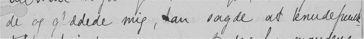de og glædede mig, han sagde at knudepunk-
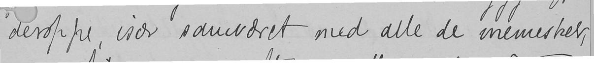deroppe, især samværet med alle de mennesker,
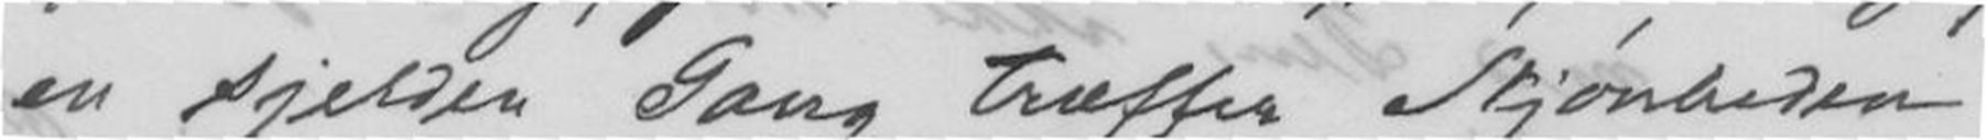en sjelden Gang træffer Skjønheden
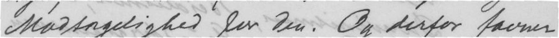Modtagelighed for den. Og derfor favner
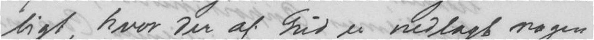ligt, hvor der af Gud er nedlagt nogen
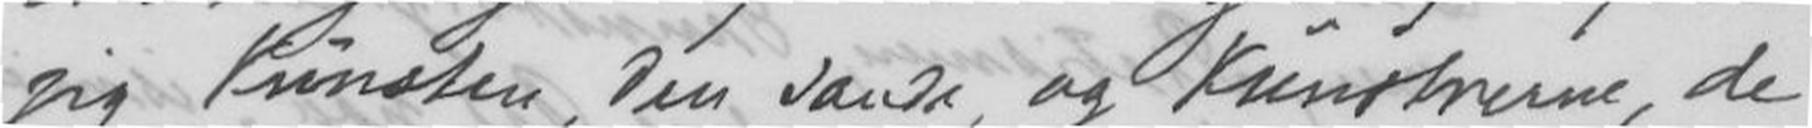jeg Kunsten, den sande, og Kunstnerne, de
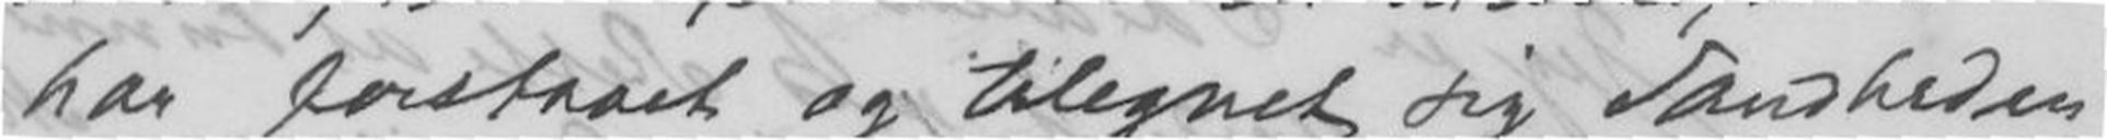har forstaaet og tilegnet sig Sandheden
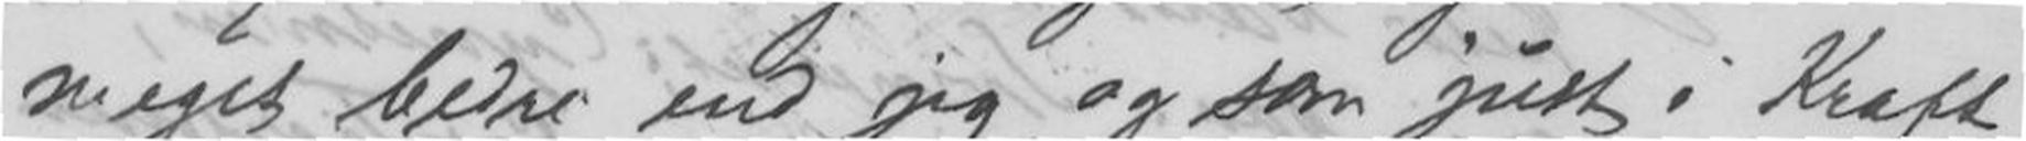meget bedre end jeg og som just i Kraft
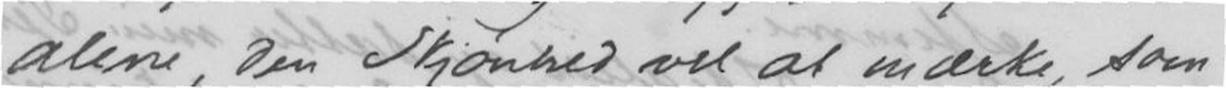alene, den Skjønhed vel at mærke, som
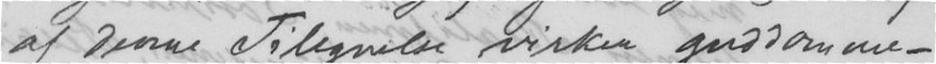af denne Tilegnelse virker guddomme-
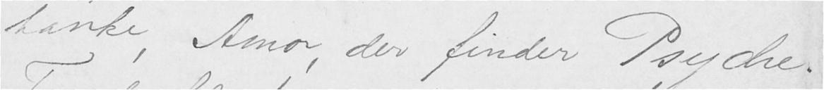tanke, Amor, der finder Psyche.
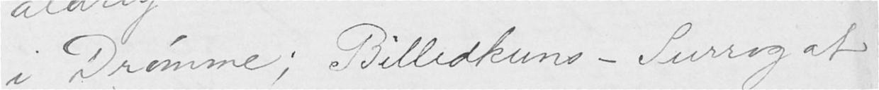i Drømme; Billedkuns — Surrogat
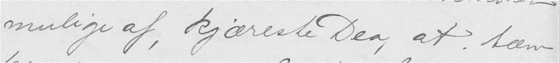mulige af, kjæreste Dea, at tæn-
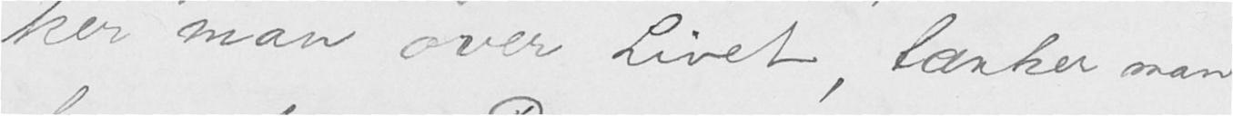ker man over Livet, tænker man
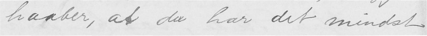haaber, at du har det mindst
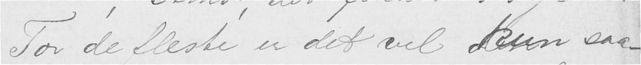For de fleste er det vel kun saa-
